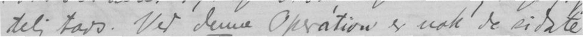telig tavs. Ved denne Opration er nok de sidste
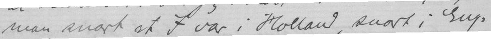man snart at I var i Holland, snart i Eng
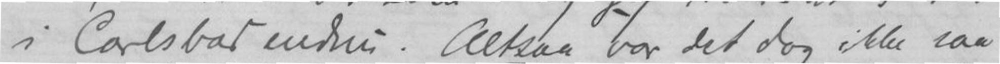i Carlstad endnu. Altsaa var det dog ikke saa
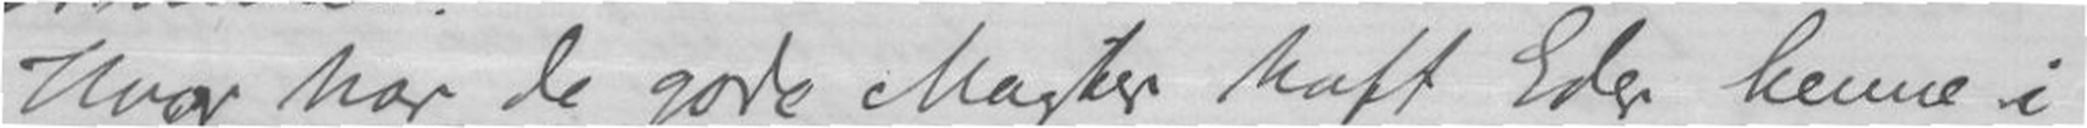Hvor har de gode Magter haft Eder henne i
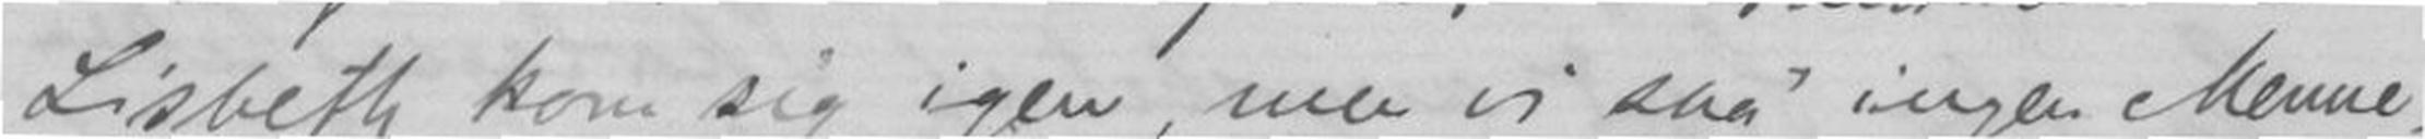Lisbeth kom sig igen, men vi saa ingen Menne-
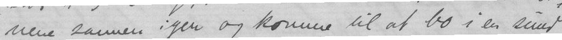nene sammen igen og komme til at bo i en sund
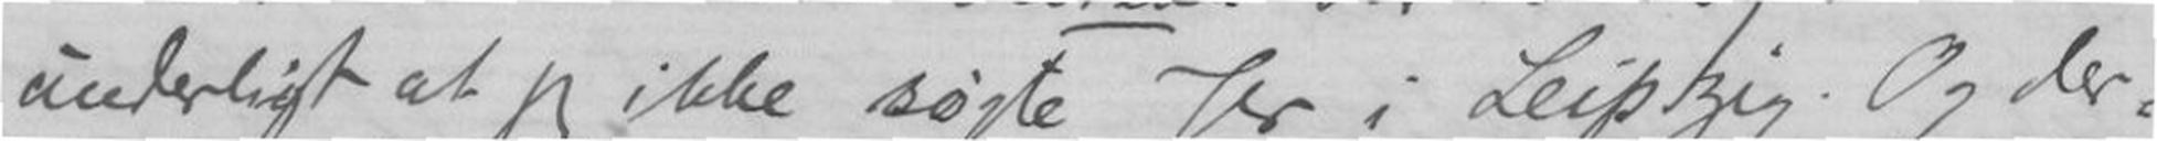underligt at jeg ikke søgte Jer i Leipzig. Og der-
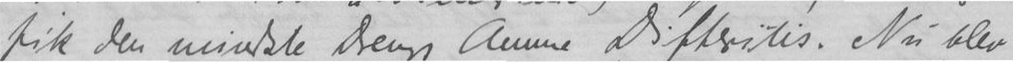fik den mindste Drengs Amme? Difteritis. Nu blev
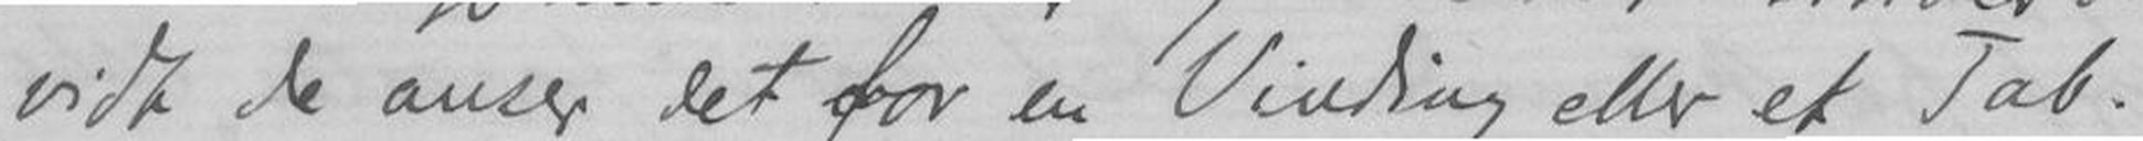vidt de anser det for en Vinding eller et Tab.
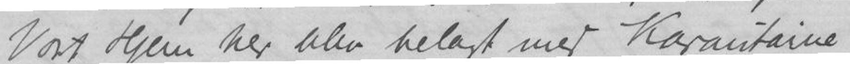Vort Hjem her blev belagt med Karantaine
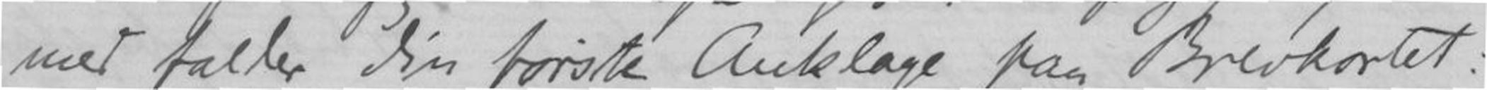med faller din første Anklage paa Brevkortet:
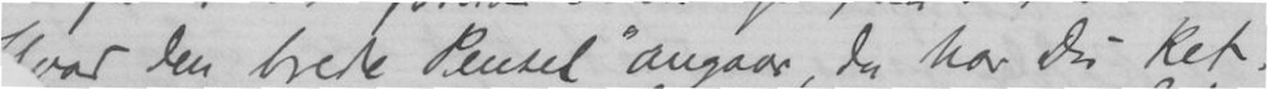Hvad den brede Pensel angaar, da har Du Ret.
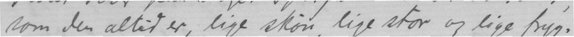som den altid er, lige stor og lige fryg-
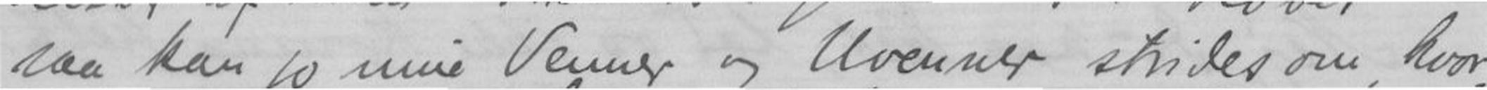saa kan jo mine Venner og Uvenner strides om, hvor
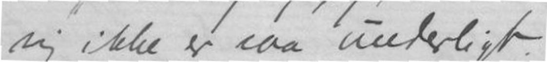sig ikke er saa underligt.
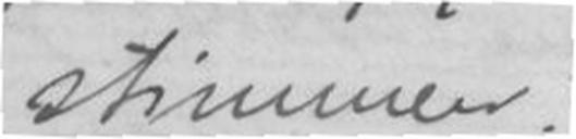stimmen!"
